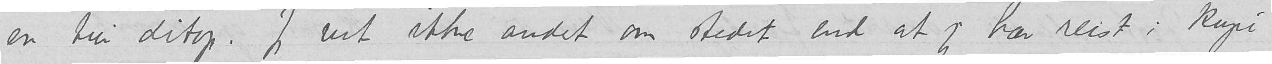en tur ditop. Jeg vet ikke andet om stedet end at jeg har reist i kupé
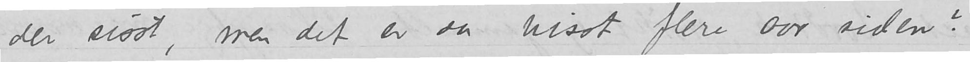der sisst, men det er da visst flere aar siden?
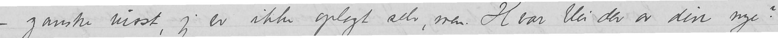– ganske visst, jeg er ikke oplagt selv, men. Hvor blir der av din nye?
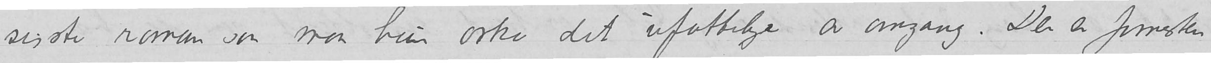sisste roman saa maa hun orke det ufattelige av omgang. Der er forresten
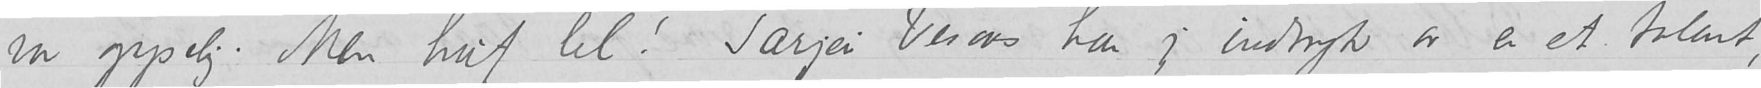var gyselig. Men huf lel! Tarjei Vesaas har jeg indtryk av er et talent,
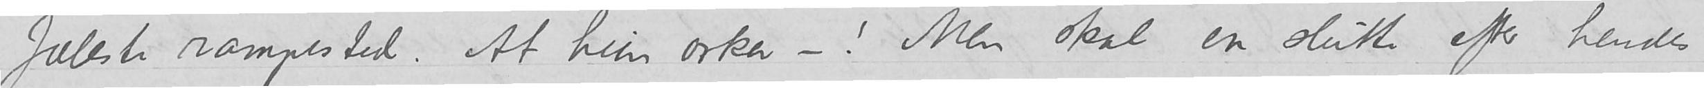fæleste rampested. At hun orker –! Men skal en slutte efter hendes
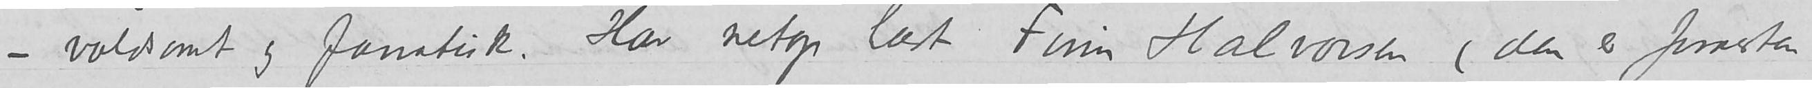– voldsomt og fanatisk. Har netop læst Finn Halvorsen (den er forresten
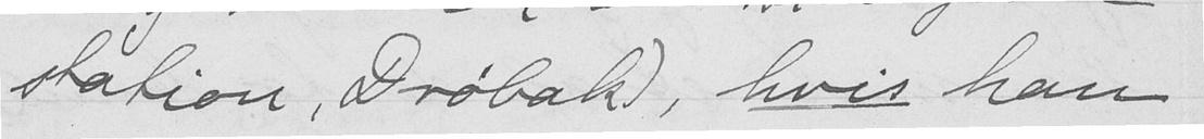station, Drøbak), hvis han
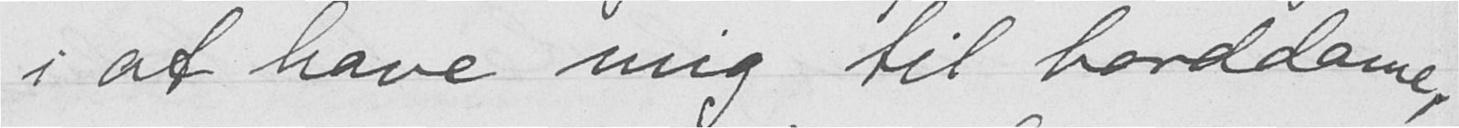i at have mig til borddame
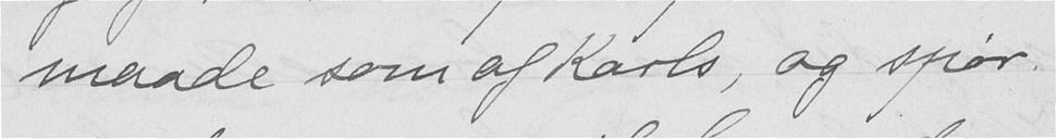maade som af Karls, og spør
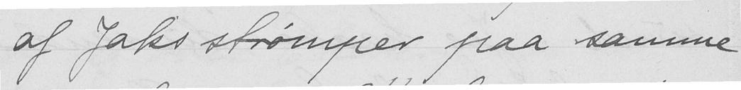af Jaks strømper paa samme
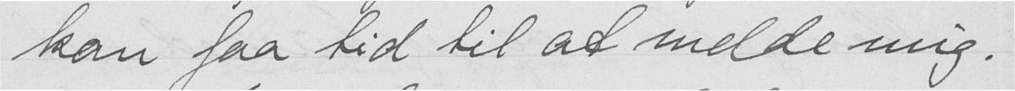kan faa tid til at melde mig.
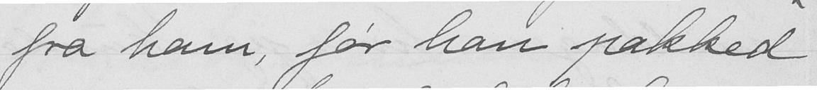fra ham, før han pakked
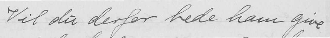Vil du derfor bede ham give
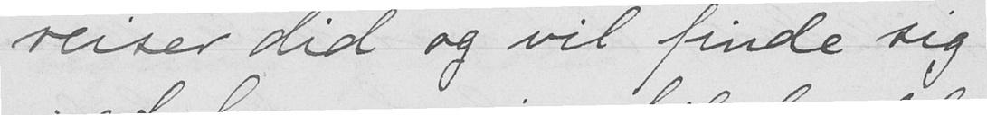reiser did og vil finde sig
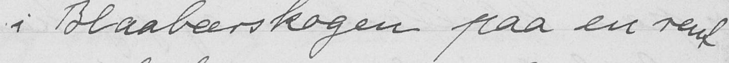i Blaabærskogen paa en rent
